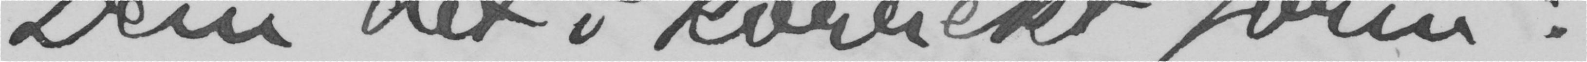Dem det i korrekt form:
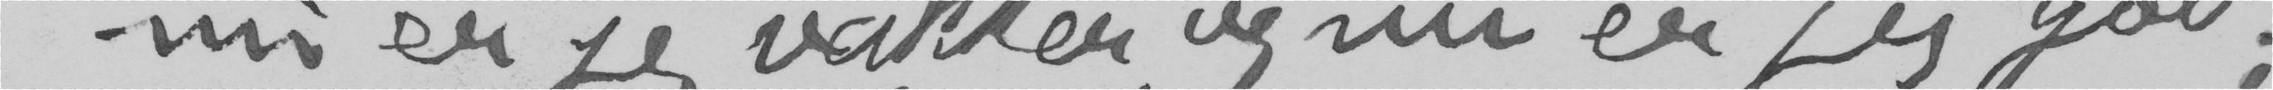nu er jeg vakker, og nu er jeg god;
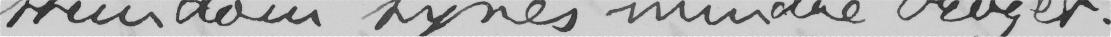stundom synes mindre broget.»
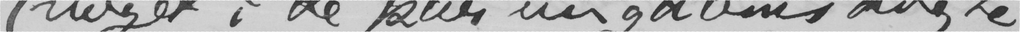noget i de par ungdomsdigte
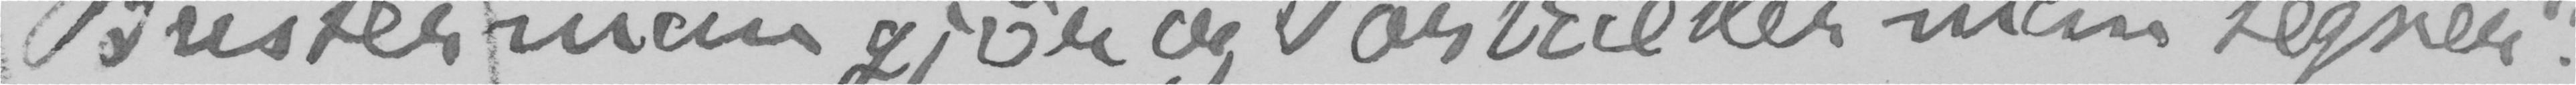Buster man gjør, og Portrætter man tegner».
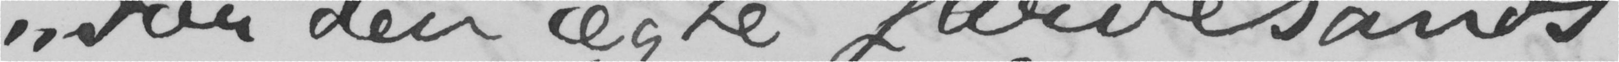«For den ægte farvesands,
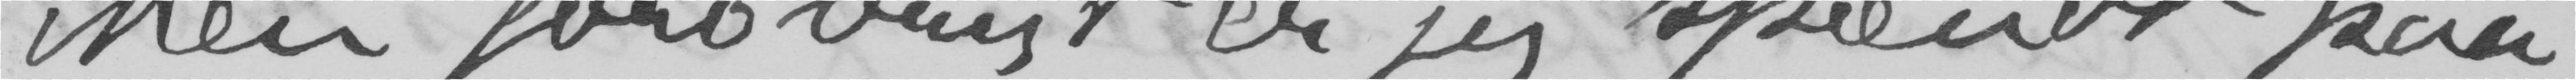Men forøvrigt er jeg spendt paa
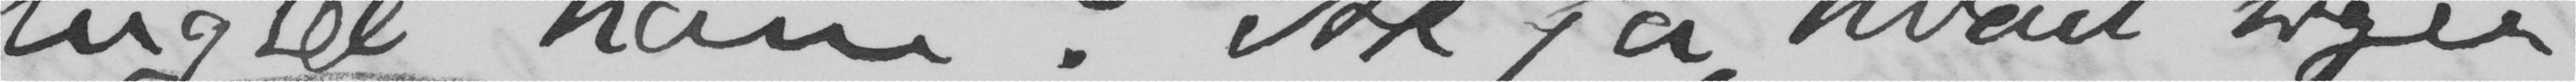lugtet ham? Ak ja, hvad siger
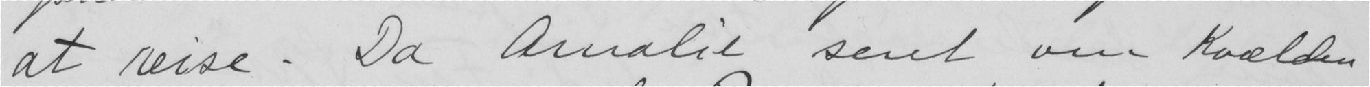at reise. Da Amalie sent om Kvælden
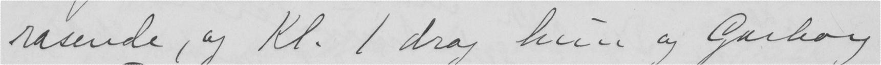rasende, og Kl. 1 drog hun og Garborg
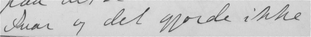Svar og det gjorde ikke
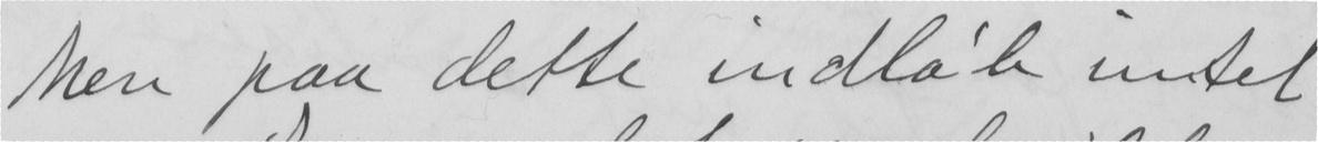Men paa dette indløb intet
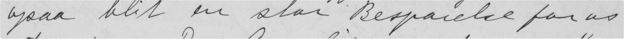ogsaa blit en stor Besparelse for os
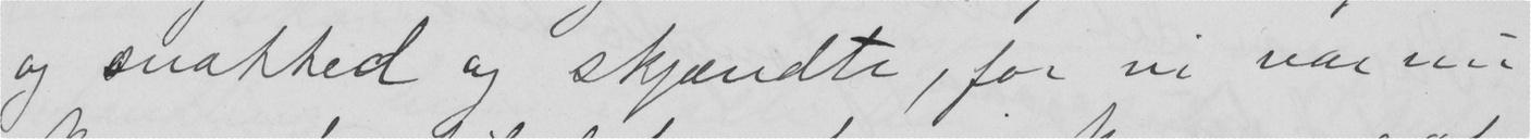og snakhed og skjendte, for vi var nu
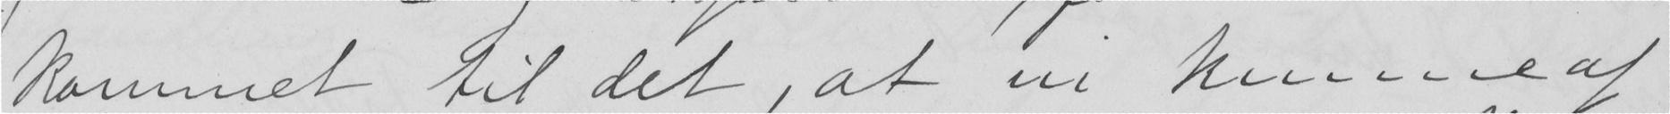kommet til det, at vi kunne af
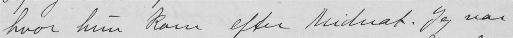hvor hun kom efter Midnat. Jeg var
